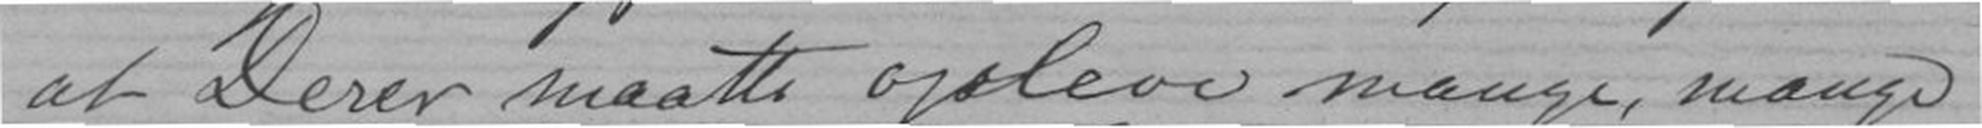at Deres maatte opleve mange, mange
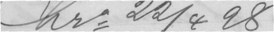Chr. 22/4 98
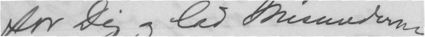for Dig og lad Mesunderne
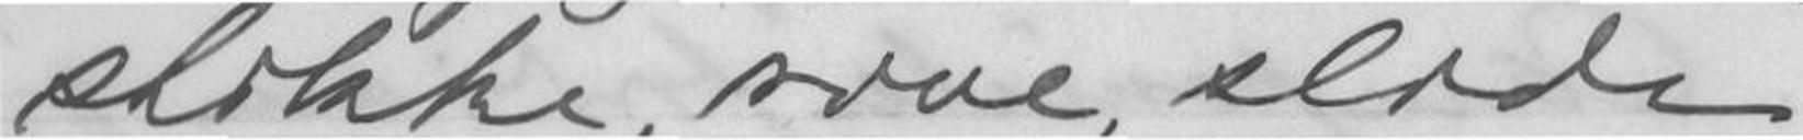slikke, sine, slide
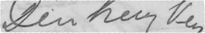Din heng Ven
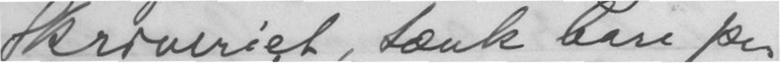Skriveriet, tænk bare paa
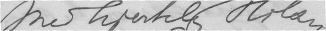Med hjertelig Hilsen
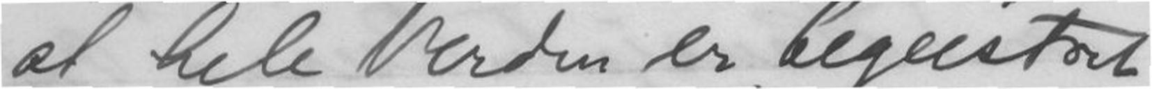at hele Verden er begeistret
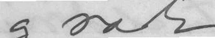og rode.
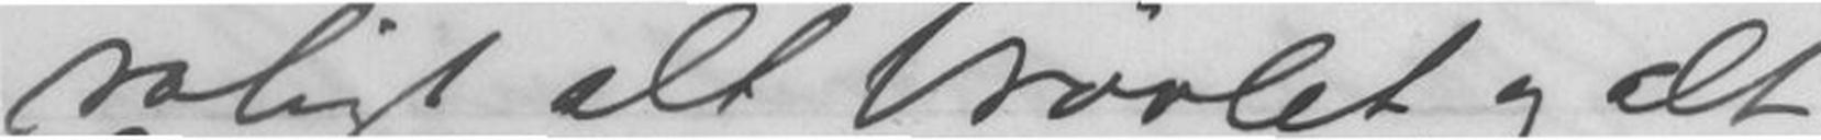roligt alt Vrøvlet og alt
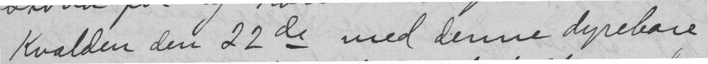Kvælden den 22de med denne dyrebare
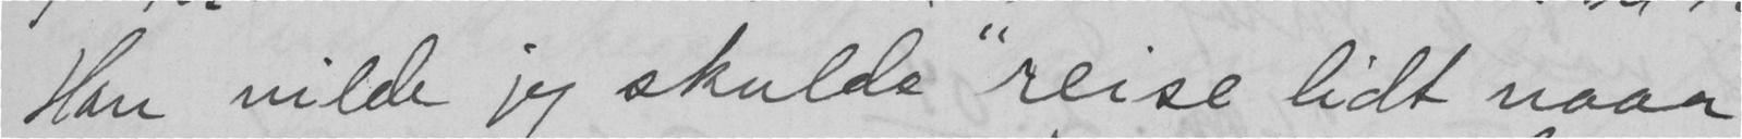Han vilde jeg skulde "reise lidt naar
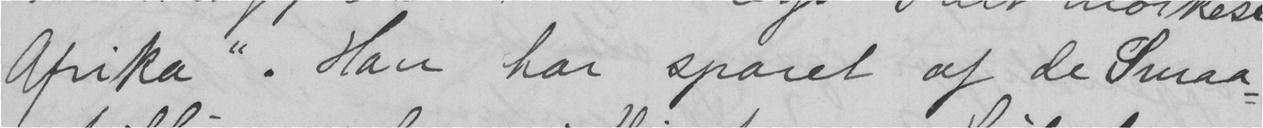Afrika". Han har sparet af de Smaa-
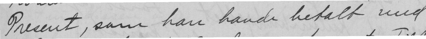Present, som han havde betalt med
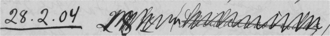28.2.04
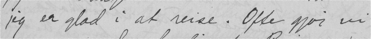jeg er glad i at reise. Ofte gjør vi
